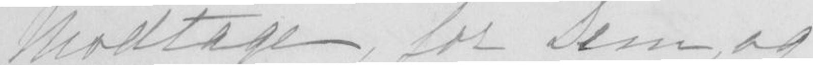Modtage, for Dem, og
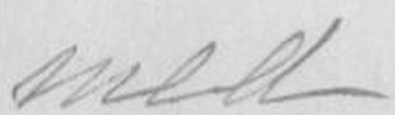med
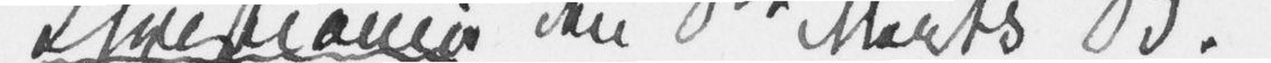Christiania den 8de Marts 85.
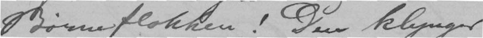Børneflokken! Den klynger
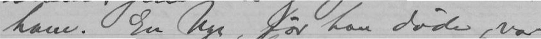ham. En Uge, før han døde, var
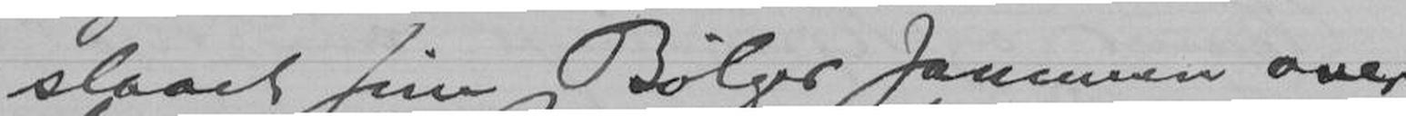slaaet sine Bølger sammen over
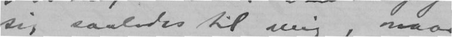sig saaledes til mig, naar
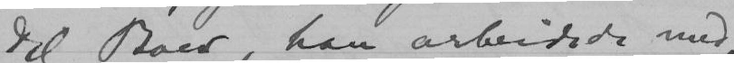Del Brev, han arbeidede med,
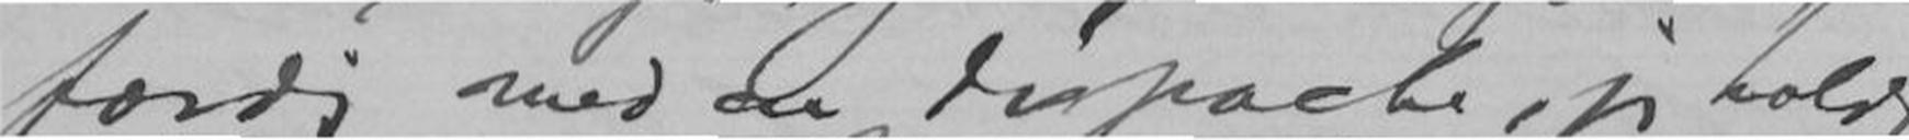færdig med en dispache, jeg holder
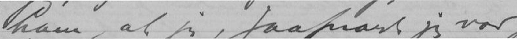ham, at jeg, saasnart jeg var
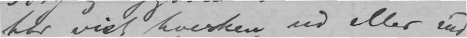har vist hverken ud eller ind,
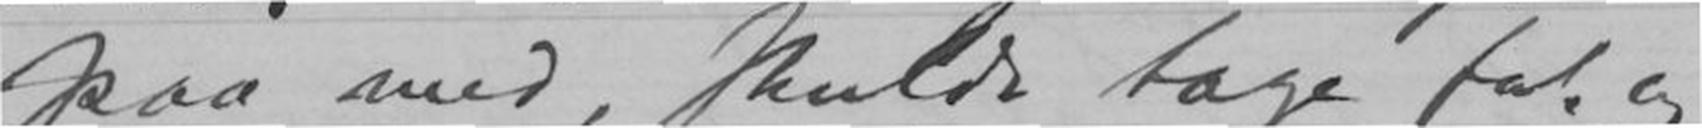paa med, skulde tage fat og
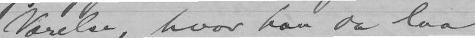Værelse, hvor han da laa
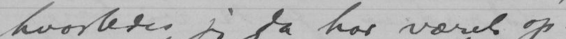hvorledes jeg da har været op-
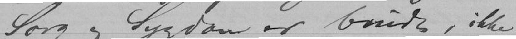Sorg og Sygdom er brudt, ikke
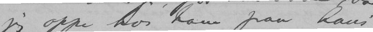jeg oppe hos ham paa hans
